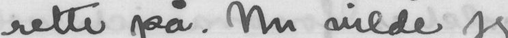rette på. Nu vilde jeg
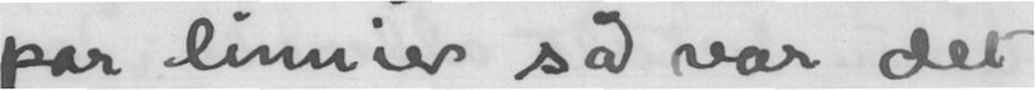par linnier så var det
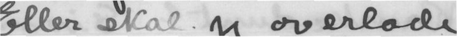Eller skal jeg overlade
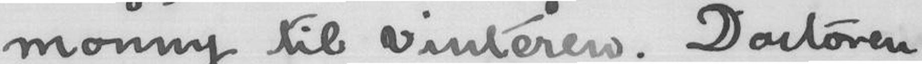monny til vinteren. Doctoren
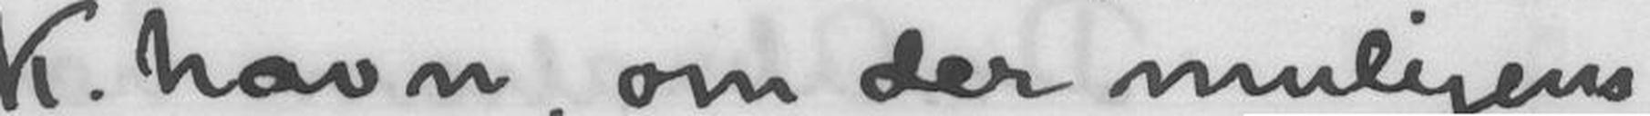K.havn, om der muligens
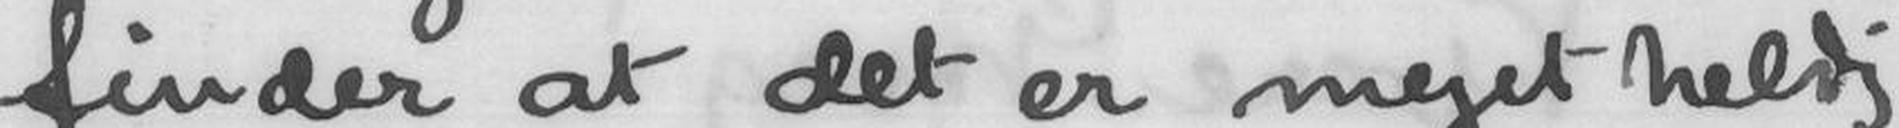finder at det er meget heldig
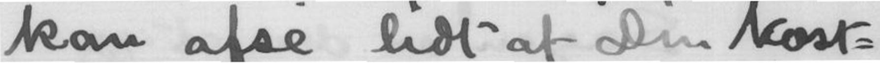kan afse lidt af Din kost-
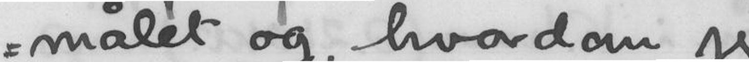målet og, hvordan jeg
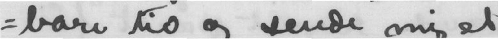bare tid og sende mig et
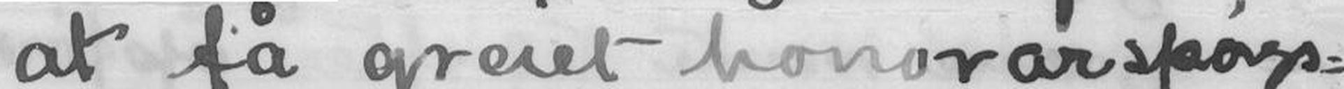at få greiet honorarspørgs-
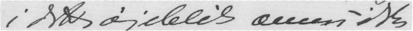i dette øjeblik ænnu ikke
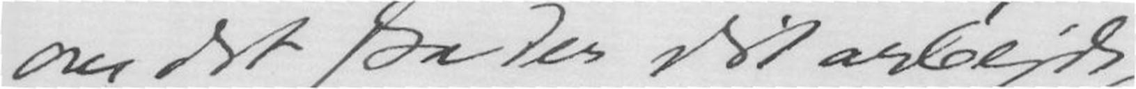om det skader dit arbejde,
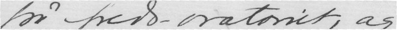på freds-oratoriet, og
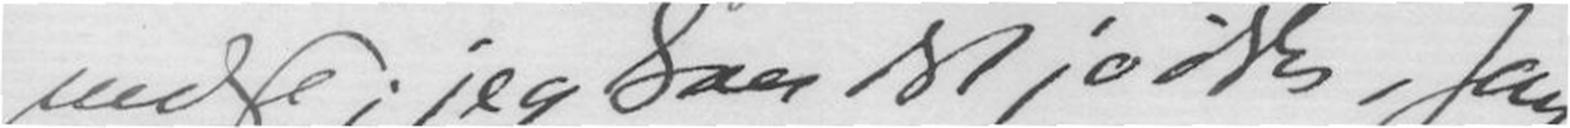melse; jeg kan det jo ikke, som
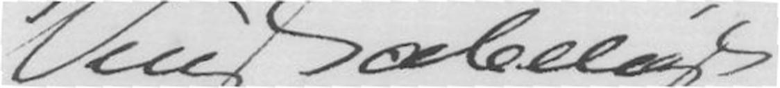Venskabeligst
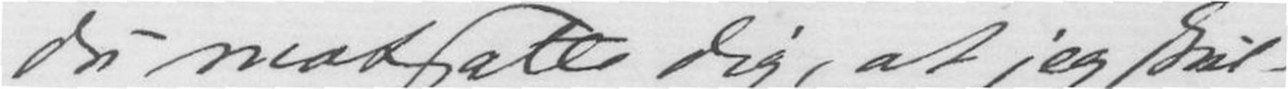Du motsatte dig, at jeg skul-
